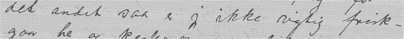det andet saa er jeg ikke rigtig frisk -
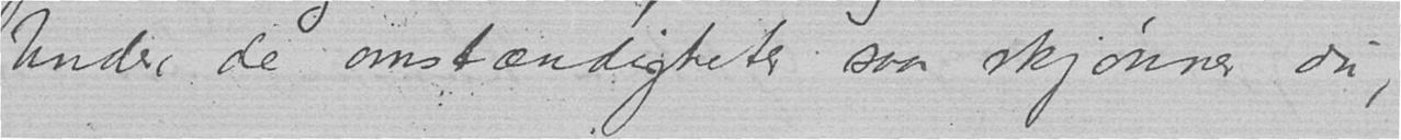Under de omstændigheter saa skjønner du,
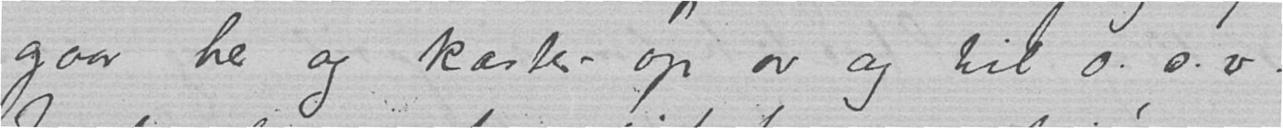gaar her og kaster op av og til o.s.v.
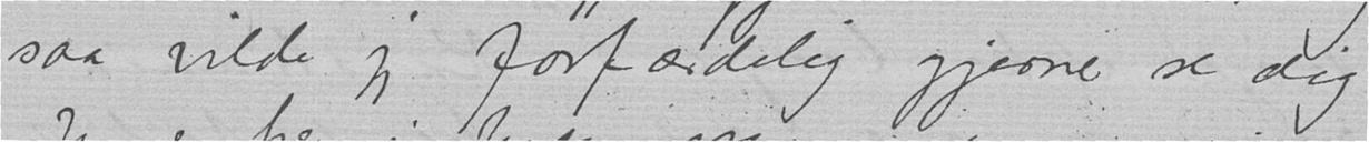saa vilde jeg forfærdelig gjerne se dig.
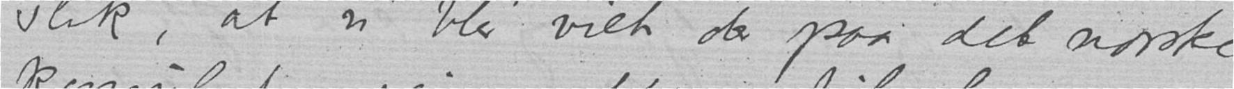slik, at vi blir viet der paa det norske
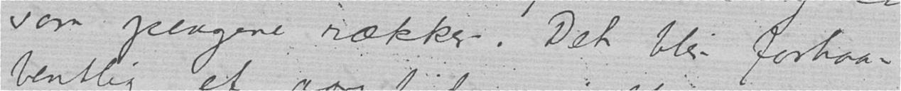som pengene rækker. Det blir forhaa-
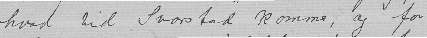hvad tid Svarstad kommer, og for
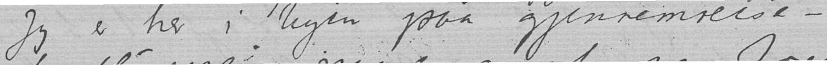Jeg er her i byen paa gjennemreise -
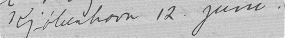Kjøbenhavn 12. juni.
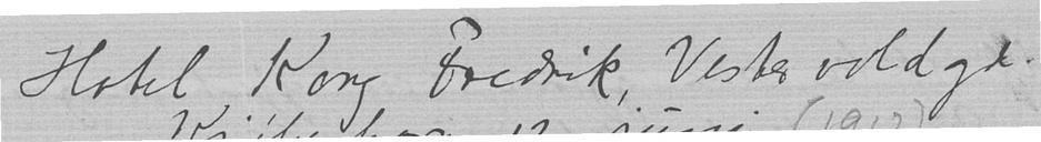Hotel Kong Fredrik, Vester voldgt.
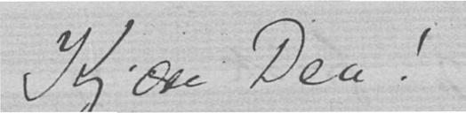Kjære Dea!
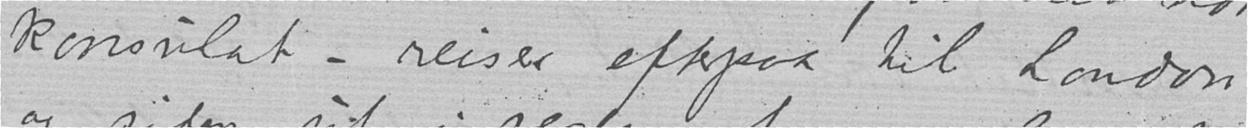konsulat - reiser efterpaa til London
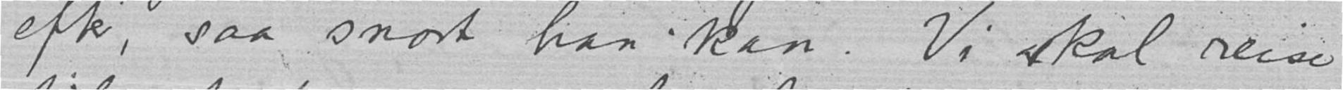efter, saa snart han kan. Vi skal reise
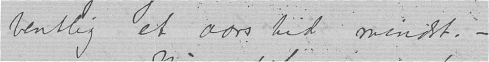bentlig et aars tid mindst. -
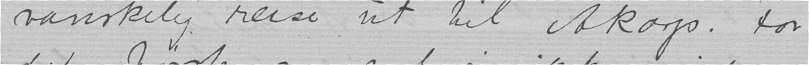vanskelig reise ut til Åkorp. For
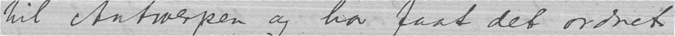til Antwerpen og har faat det ordnet
